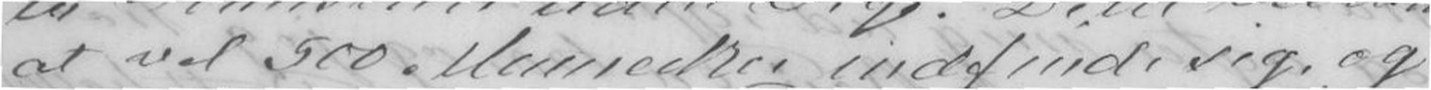at vel 500 Mennesker indfinde sig, og
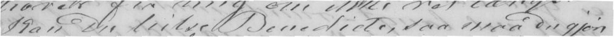Kan Du hilse Benedicte, saa maa Du gjøre
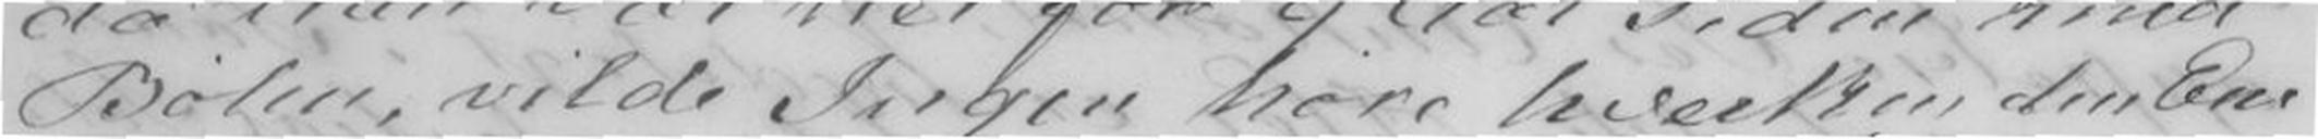Bøhn, vilde Ingen høre hverken den Ene
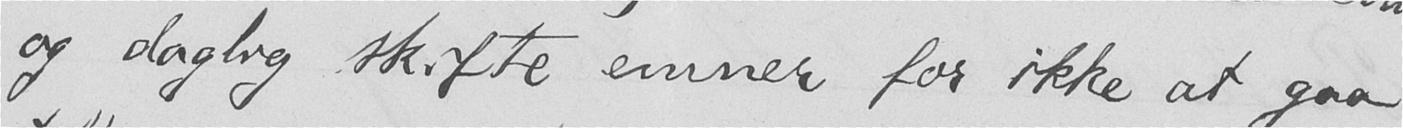og daglig skifte emner for ikke at gaa
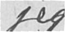jeg
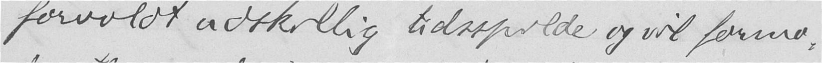forvoldt adskillig tidsspilde og vil formo-
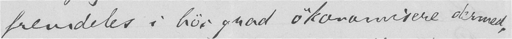fremdeles i høi grad økonomisere dermed,
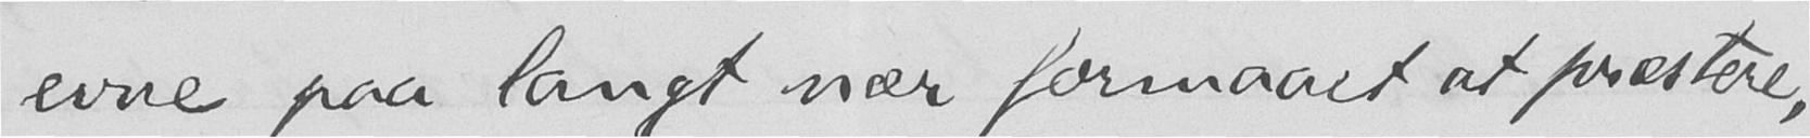evne paa langt nær formaaet at præstere,
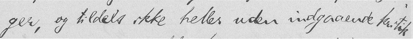ger, og tildels ikke heller uden indgaaende kritik,
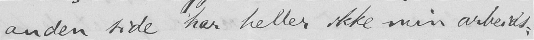anden side har heller ikke min arbeids-
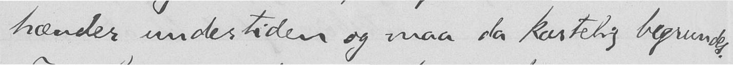hænder undertiden og maa da kortelig begrundes.
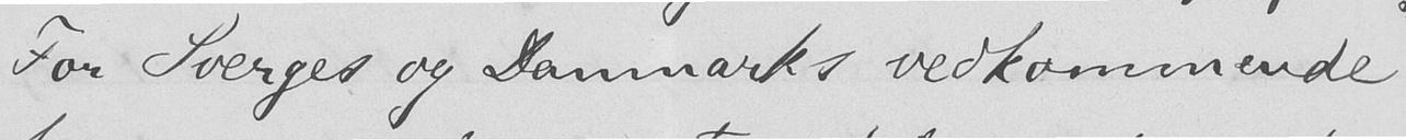For Sverges og Danmarks vedkommende
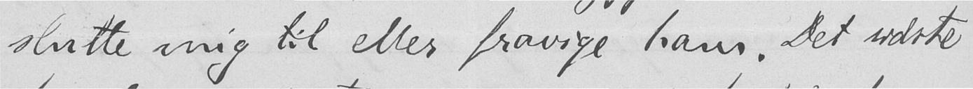slutte mig til eller fravige ham. Det sidste
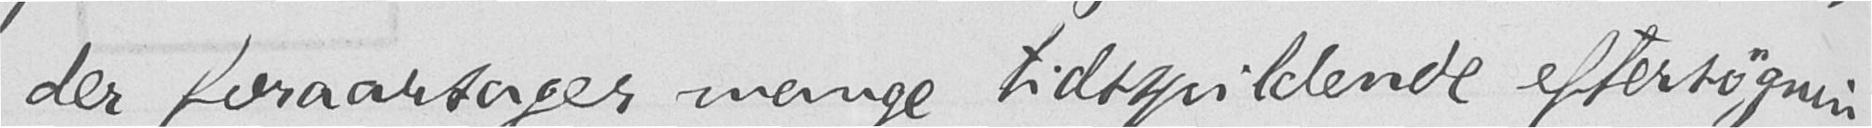der foraarsager mange tidsspildende eftersøgnin-
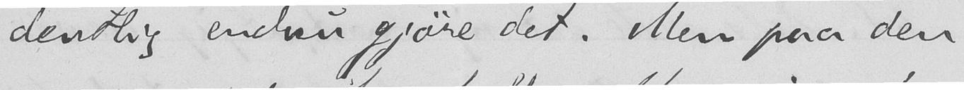dentlig endnu gjøre det. Men paa den
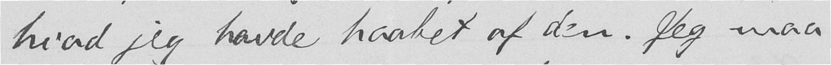hvad jeg havde haabet af den. Jeg maa
